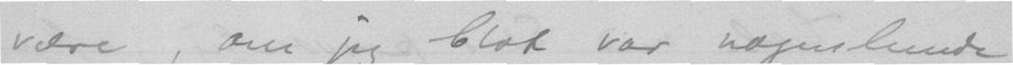være, om jeg blot var nogenlunde
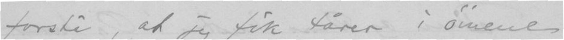forstå, at jeg fik tårer i øinene
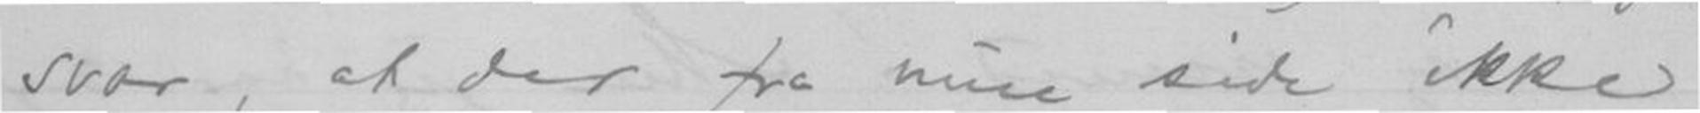svor, at der fra min side ikke
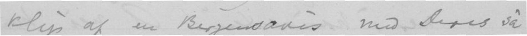klip af en Bergensavis med Deres så
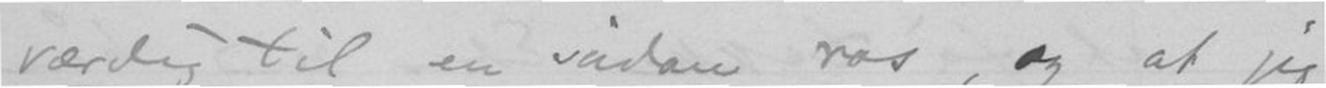værdig til en sådan ros, og at jeg
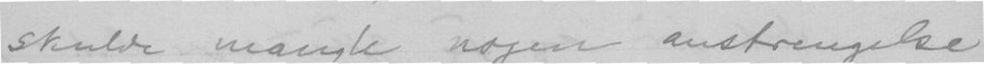skulde mangle nogen anstrengelse
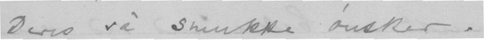Deres så smukke ønsker.
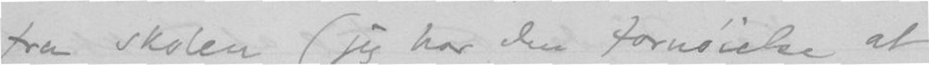fra skolen (jeg har den fornøielse at
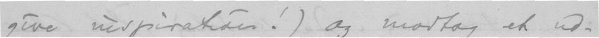give inspiration!) og modtog et ud-
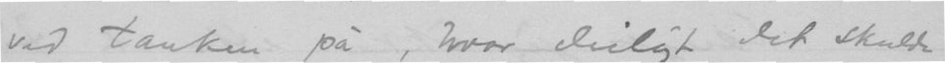ved tanken på, hvor deiligt det skulde
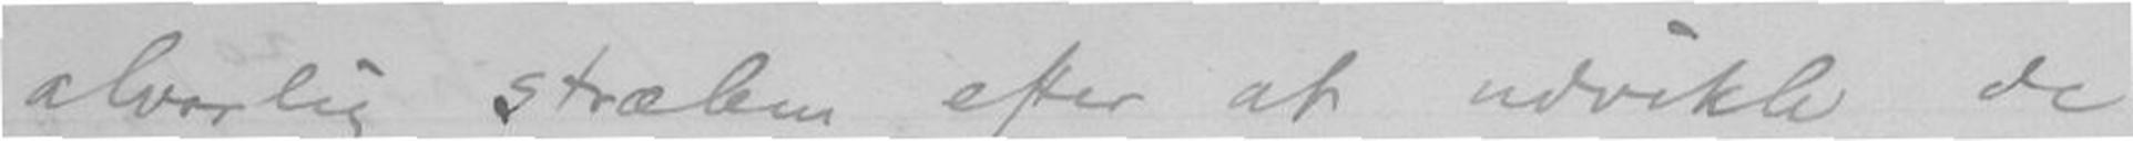alvorlig stræben efter at udvikle de
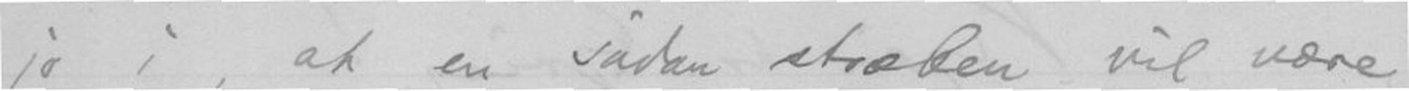jo i, at en sådan stræben vil være
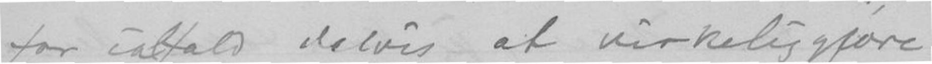for ialfald delvis at virkeliggjøre
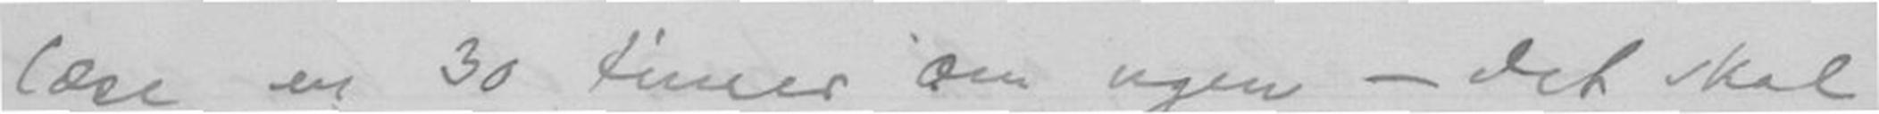læse en 30 timer om ugen - det skal
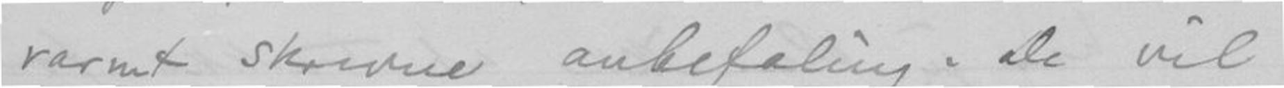varmt skrevne anbefaling. De vil
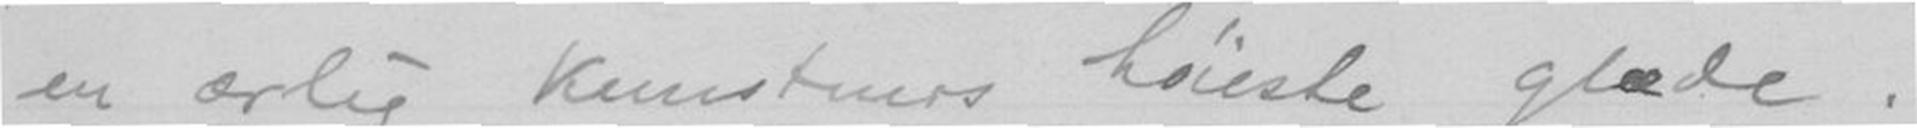en ærlig kunstners høieste glæde.
